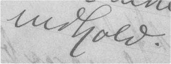indhold.)
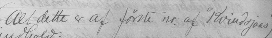(Alt dette er af første nr. af "Kvindsjaas"
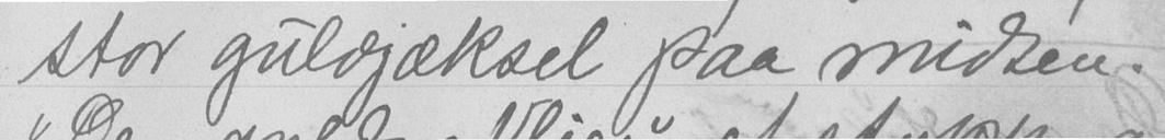stor guldjæksel paa midten.
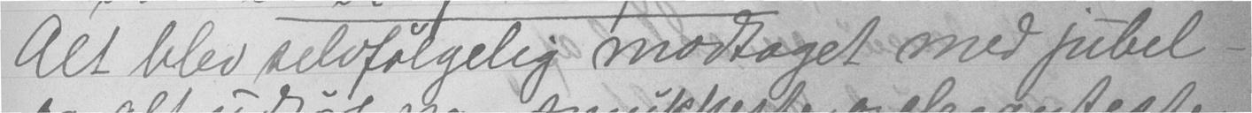Alt blev selvfølgelig modtaget med jubel -
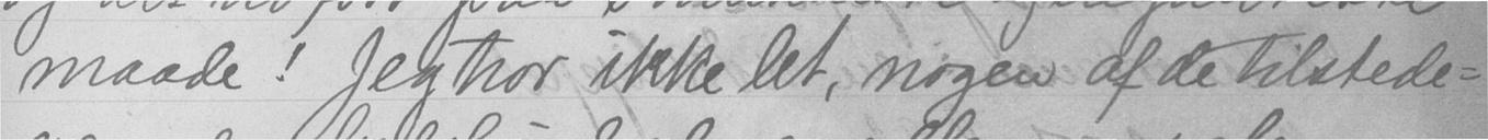maade! Jeg tror ikke let, nogen af de tilstede-
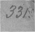331.
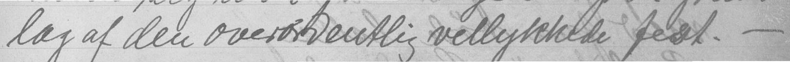lag af den overordentlig vellykkede fest. -
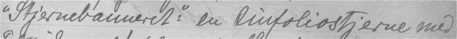"Sjernebanneret:" en tinfoliostjerne med
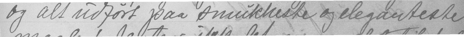og alt udført paa smukheste og eleganteste
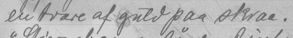en tvare af guld paa skraa.
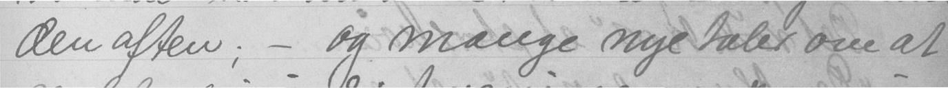den aften; - og mange nye taler om at
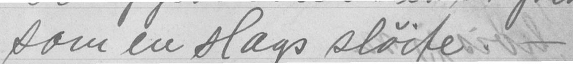som en slags sløife. -
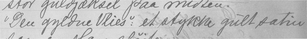"Den gyldne Vlies": et stykke gult satin
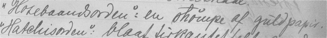"Hosebaandsorden": en shømpe af guldpapir.
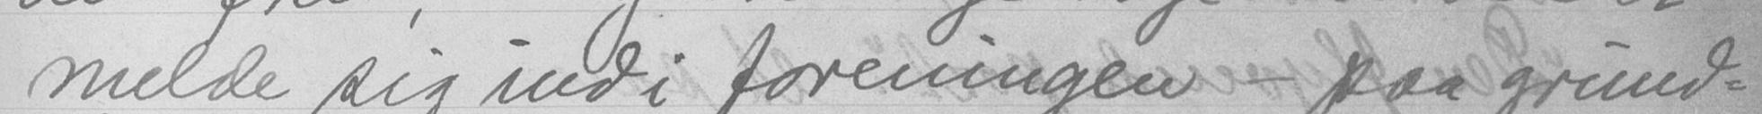melde sig ind i foreningen - paa grund-
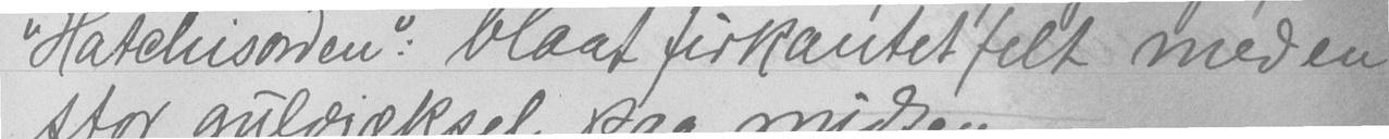"Hatchisorden: blaat firkantet felt med en
